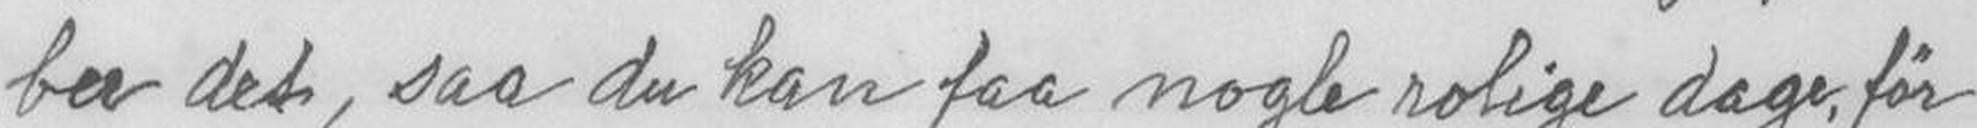ber det, saa du kan faa nogle rolige dager, før
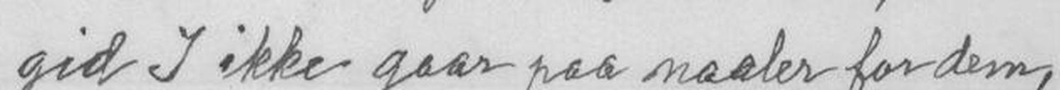gid I ikke gaar paa maaler for dem for dem,
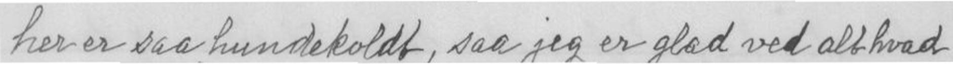her er saa hundekoldt, saa jeg er glæd ved alt hvad
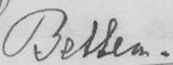Betten.
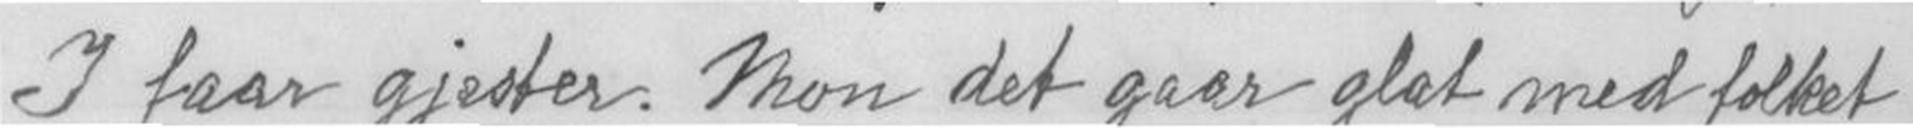I faar gjester. Mon det gaar glat med folket
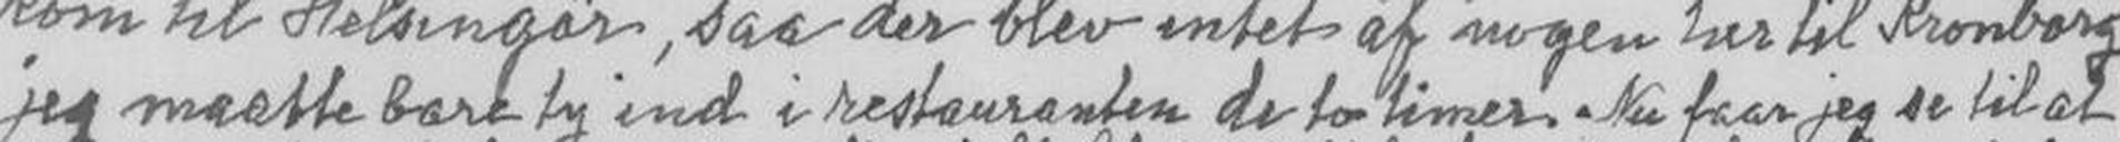jeg maatte bare ty ind i restauranten der de to timer. Nu faar jeg se til at
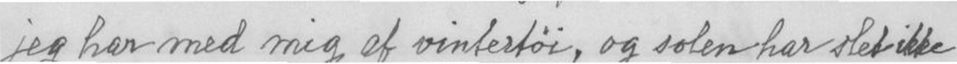jeg har med mig af vintertøi, og solen har Net ikke
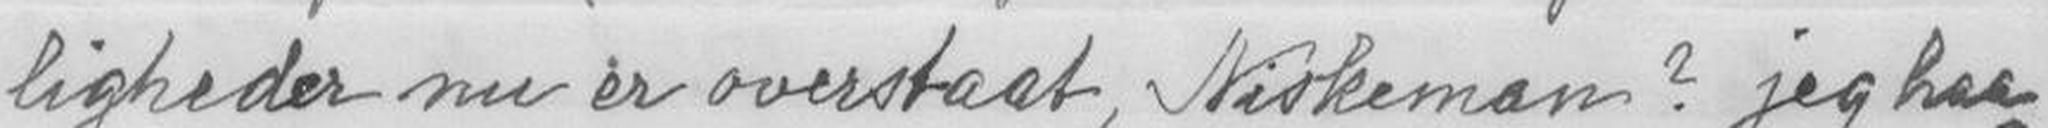ligheder nu er overstaat, Niskeman? jeg haa-
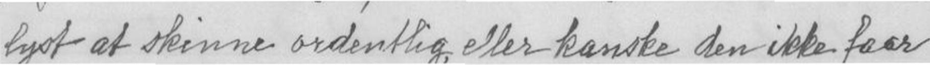lyst at skinne ordentlig, eller kanske den ikke faar
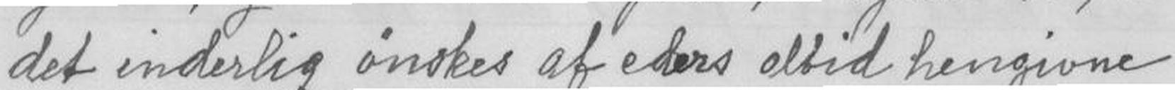det inderlig ønskes af eders altid hengivne
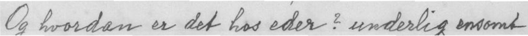Og hvordan er det hos eder? underlig ensomt
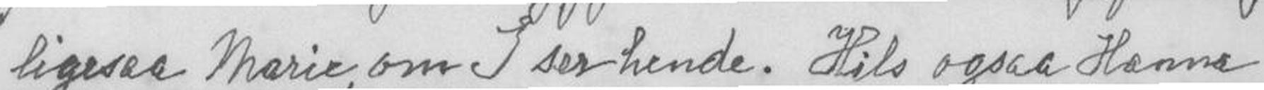ligesaa Marie, om I ser hende. Hils ogsaa Hanna
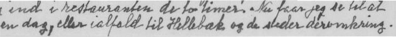en dag, eller ialfald til Hellebak og da steder deromkring.
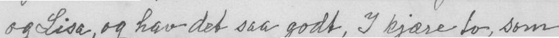og Lisa, og hav det saa godt, I kjære to, som
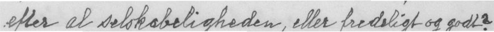efter al selskabeligheden, eller fredeligt og godt?
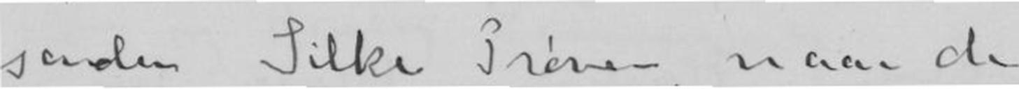sender Silke Prøver, naar de
